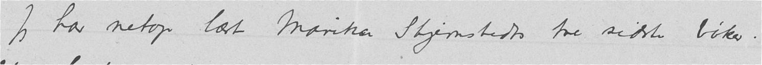Jeg har netop læst Marika Stjernstedts tre sidste bøker.
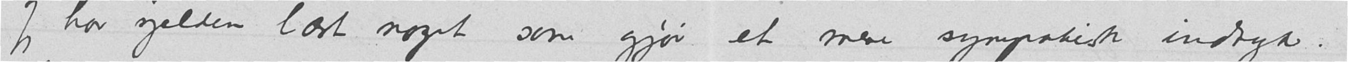Jeg har sjelden læst noget som gjør et mere sympatisk indryk.
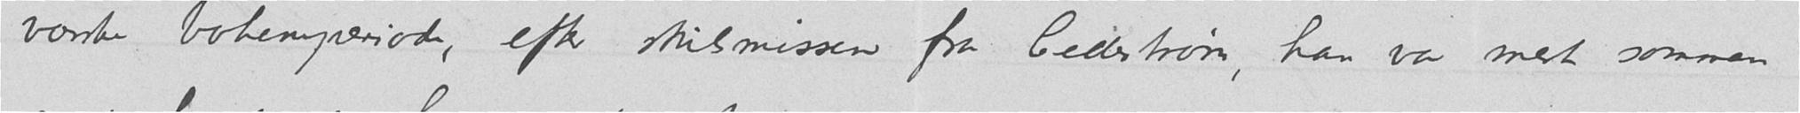værste bohemperiode, efter skilsmissen fra Cederstrøm, han var mest sammen
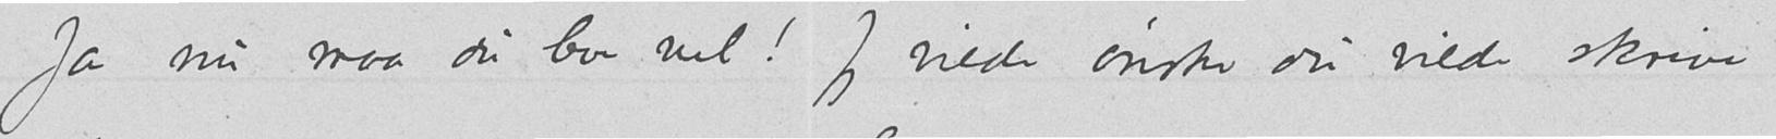Ja nu maa du leve vel! Jeg vilde ønske du vilde skrive
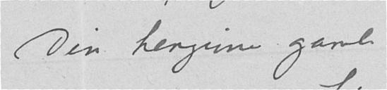Din hengivne gamle
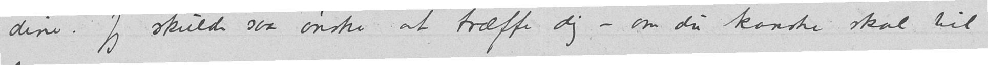dine. Jeg skulde saa ønske at træffe dig - om du kanske skal til
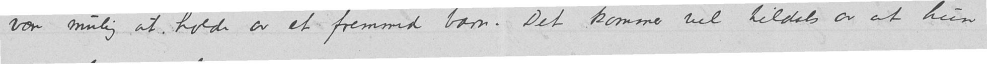være mulig at holde av et fremmed barn. Det kommer vel tildels av at hun
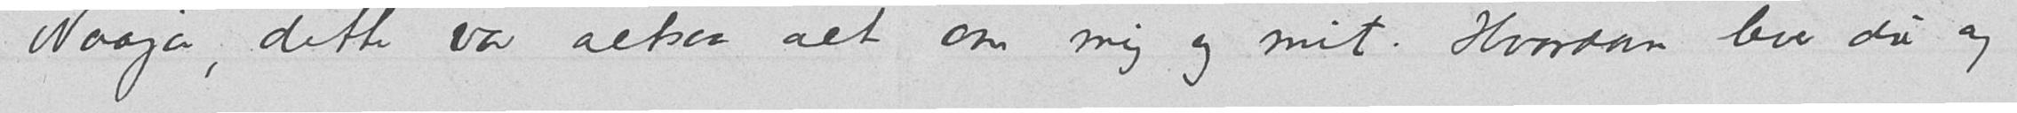Naaja; dette var altsaa alt om mig og mit. Hvordan lever du og
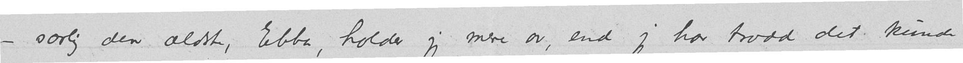- særlig den ældste, Ebba, holder jeg mere av, end jeg har trodd det kunde
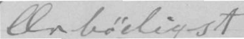Ærbødigst
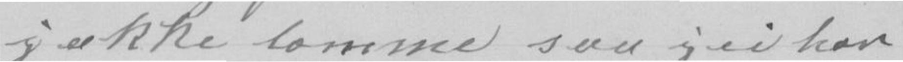jakke lomme saa jei har
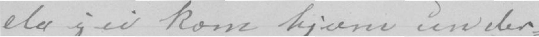da jei kom hjæm under-
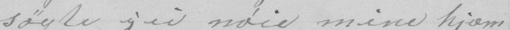søgte jei nøie mine hjæm-
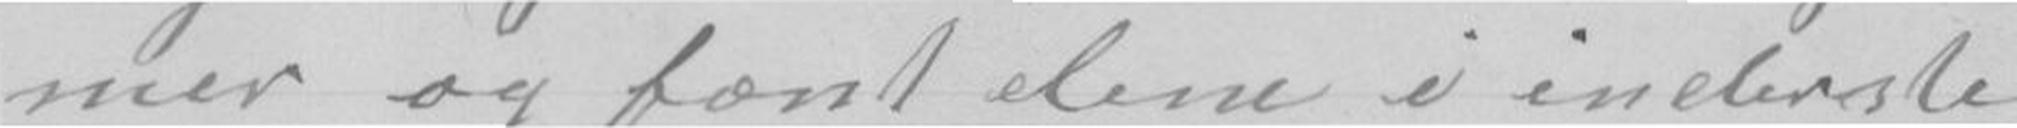mer og font dem i inderste
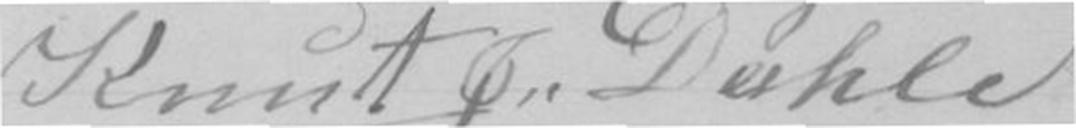Knut J. Dahle
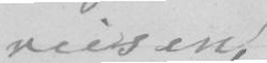reisen,
